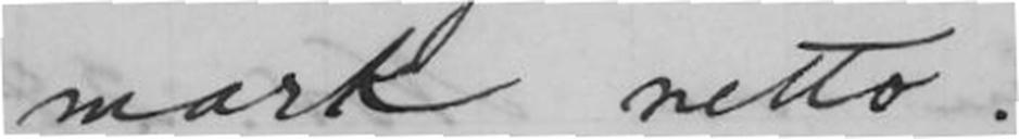mark netto.
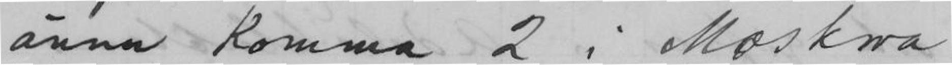ännu komma 2 i Moskwa
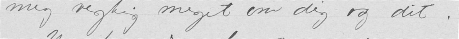mig rigtig meget om dig og dit.
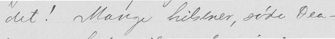det! Mange hilsener, søde Dea -
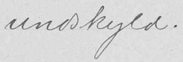undskyld.
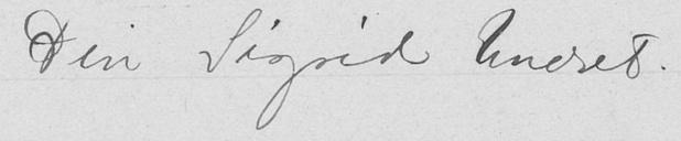Din Sigrid Undset.
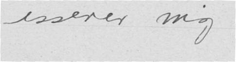esserer mig
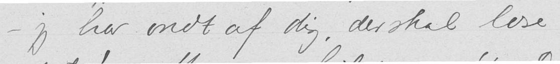- jeg har ondt af dig, der skal læse
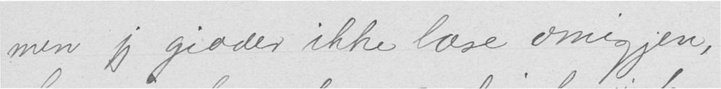min jeg gidder ikke læse omigjen,
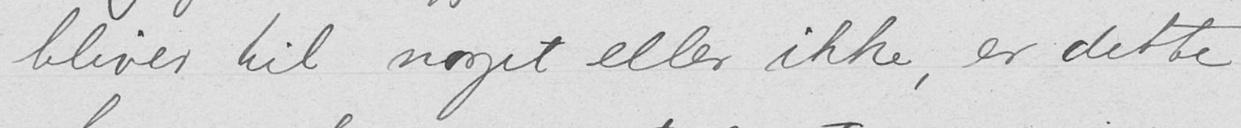bliver til noget eller ikke, er dette
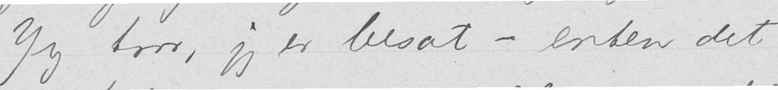Jeg tror, jeg er besat - enten det
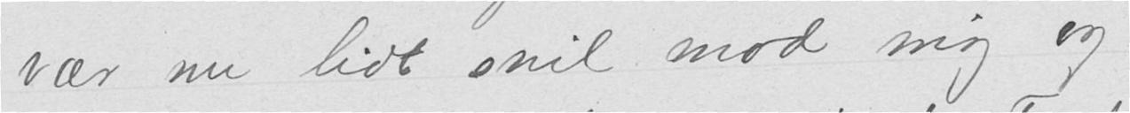vær nu lidt snil mod mig og
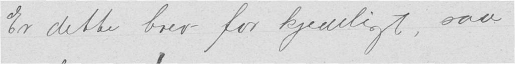Er dette brev for kjedeligt, saa
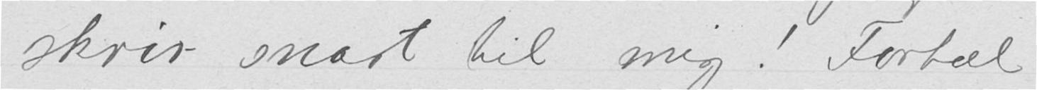skriv snart til mig! Fortæl
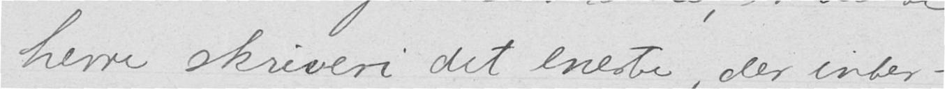herre skriveri det eneste, der inter-
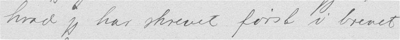hvad jeg har skrevet først i brevet
